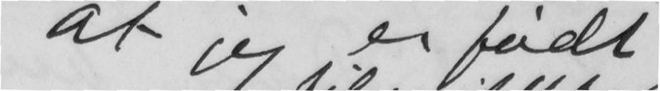at er født
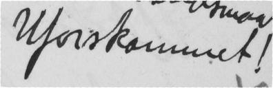Uforskammet!
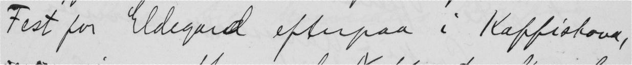Fest for Eldegard efterpaa i Kaffistova,
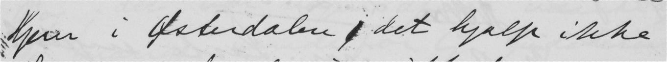Hjem i Østerdalen, det hjalp ikke
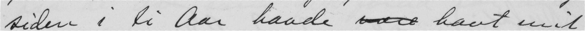siden i ti Aar havde  havt mit
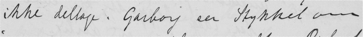ikke deltage. Garborg ser Stykket om
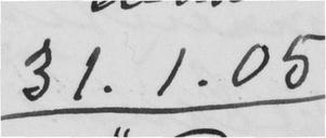31.1.05
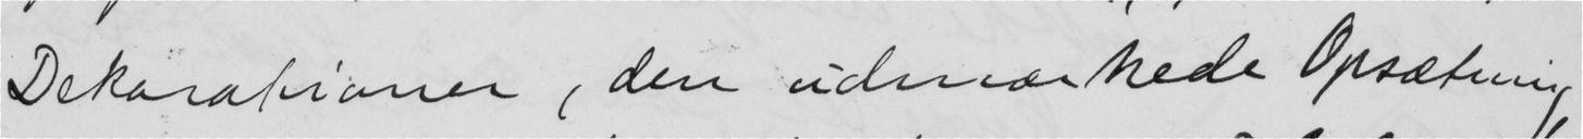Dekorationer, den udmærkede Opsætning,
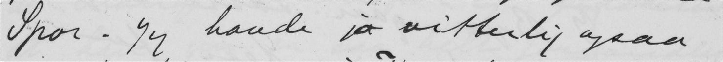Spor. Jeg havde jo vitterlig ogsaa
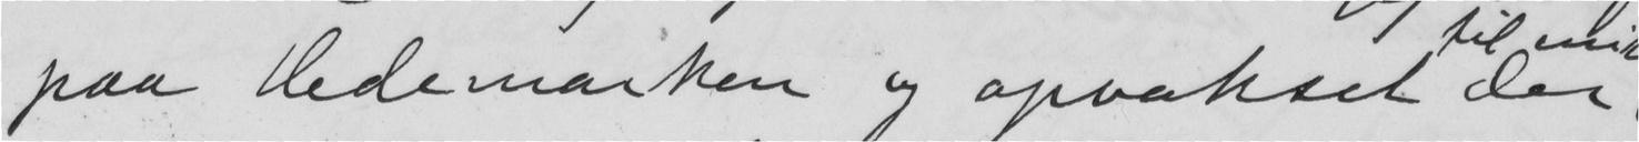paa Hedemarken og opvokset der
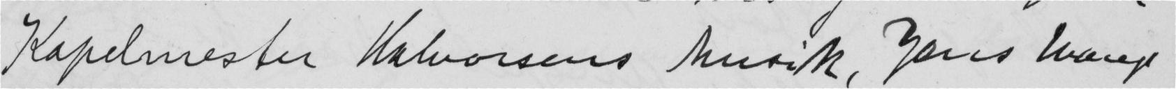Kapelmester Halvorsens Musik, Jens Wange
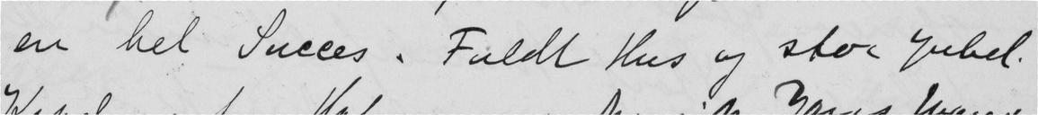en hel Succes. Fuldt Hus og stor Jubel.
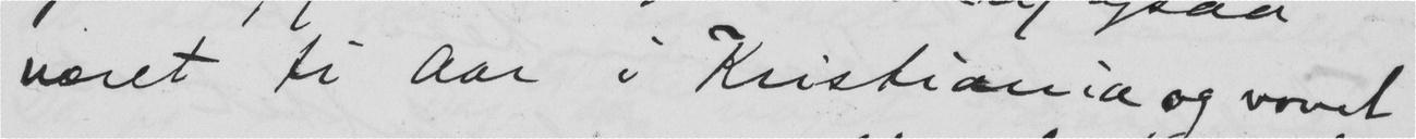været ti Aar i Kristiania og vovet
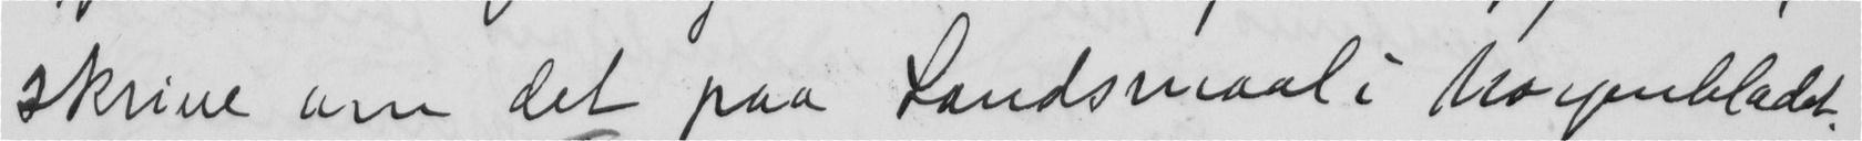skrive om det paa Landsmaal i Morgenbladet.
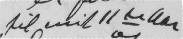til mit 11te Aar
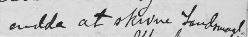endda at skrive Landsmaal
